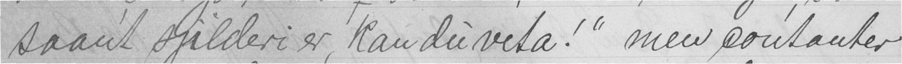saant sjilderi er, kan du veta!" men contanter
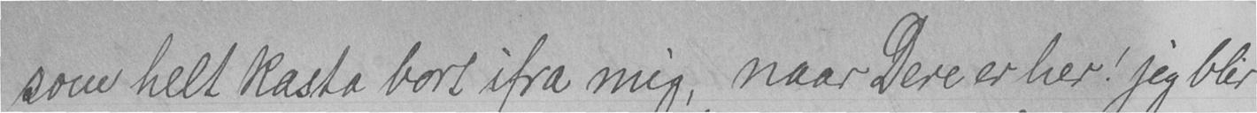som helt kasta bort ifra mig, naar Dere er her! Jeg blir
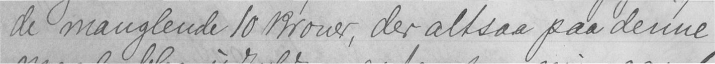de manglende 10 kroner, der altsaa paa denne
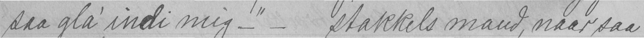saa gla' indi mig - " - stakkels mand, naar saa
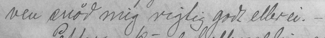ven snød mig rigtig godt eller ei. -
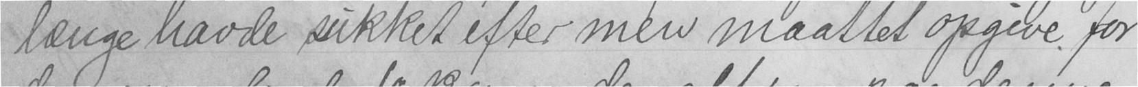længe havde sukket efter men maattet opgive. for
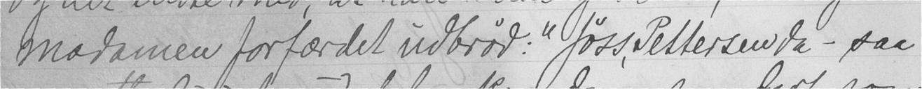madamen forfærdet udbrød: "Jøss, Pettersen da - saa
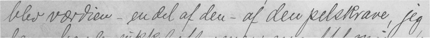blev værdien - en del af den - af den pelskrave, jeg
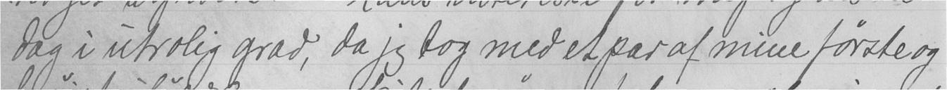dag i utrolig grad, da jeg tog med et par af mine første og
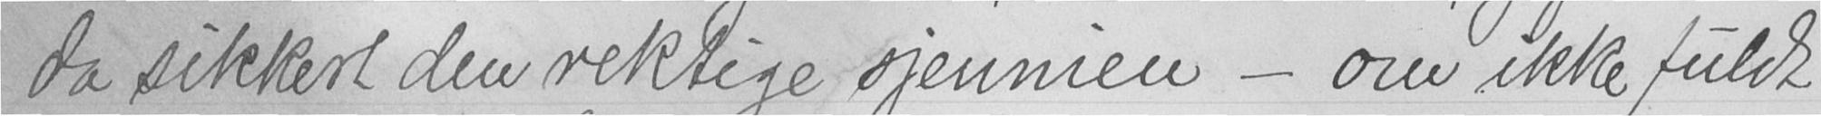da sikkert den rektige sjennien - om ikke fuldt
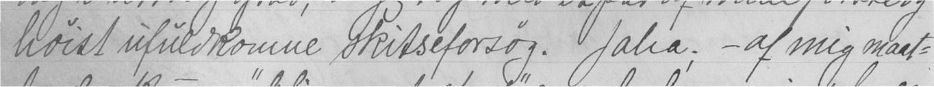høist ufuldkomne skitseforsøg. Jaha; - af mig maat-
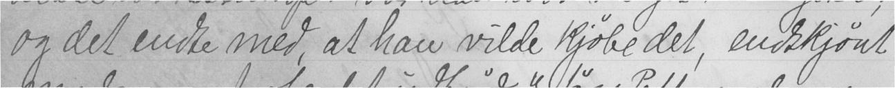og det endte med, at han vilde kjøbe det, endskjønt
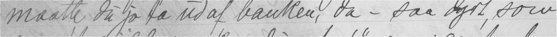maatte du jo ta ud af banken, da - saa dyrt, som
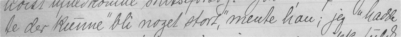te der kunne bli noget stort," mente han; jeg "hadde
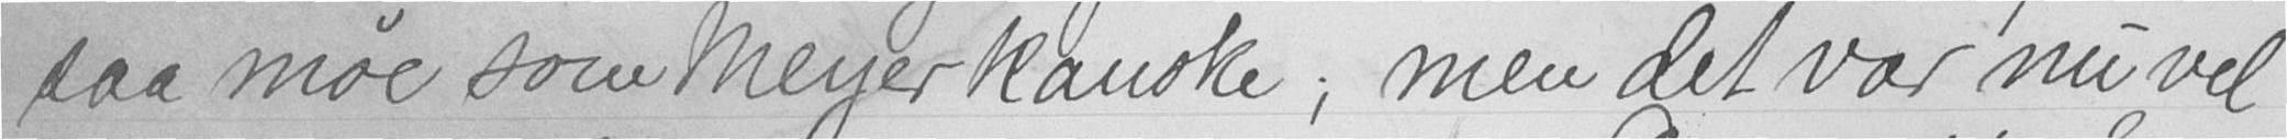saa møe som Meyer kanske; men det var nu vel
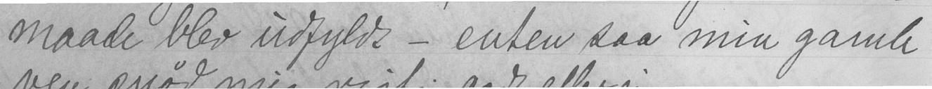maade blev udfyldt - enten saa min gamle
